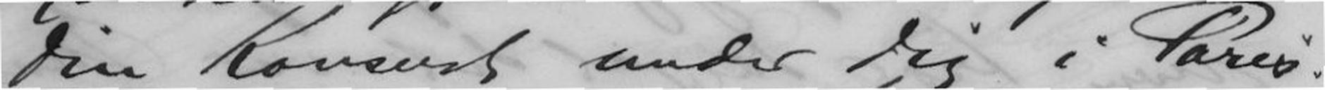Din Konsert under Dig i Paris.
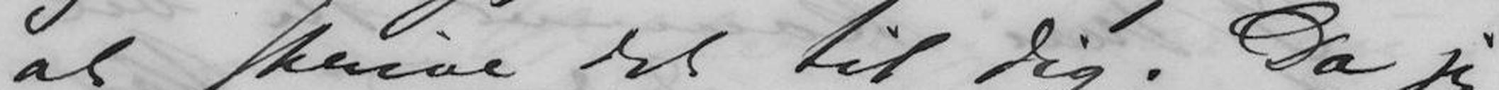at skrive det til Dig. Da jeg
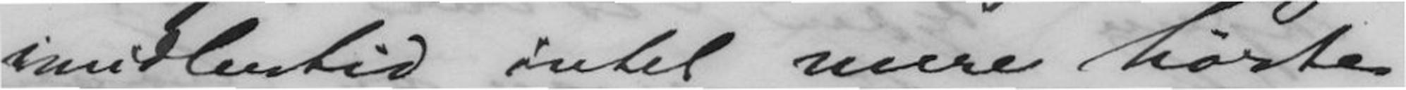imidlertid intet mere hørte
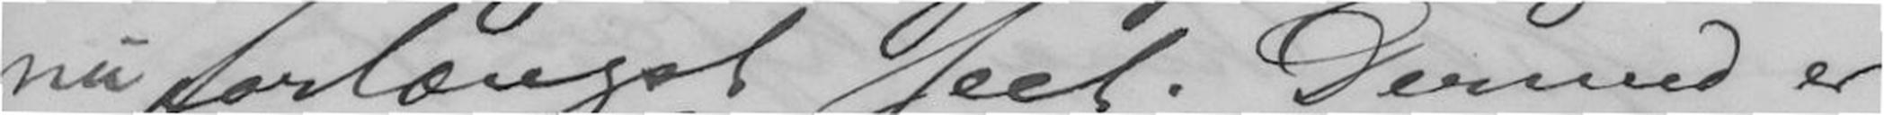nu forlængst seet. Dermed er
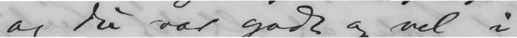og Du var godt og vel i
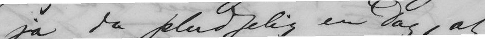jeg da pludselig en Dag, at
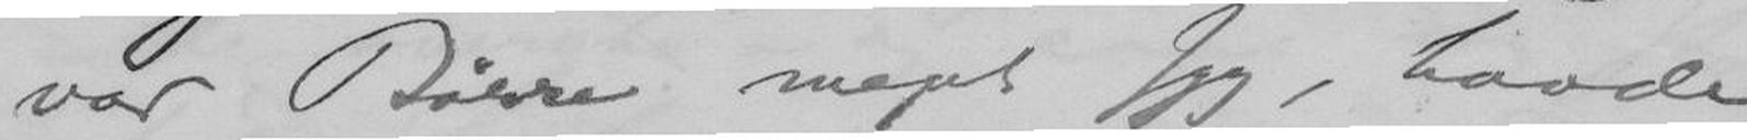var Børre meget syg, havde
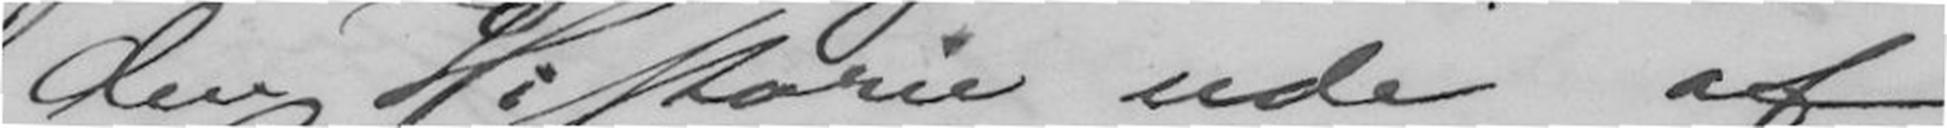den Historie ude af
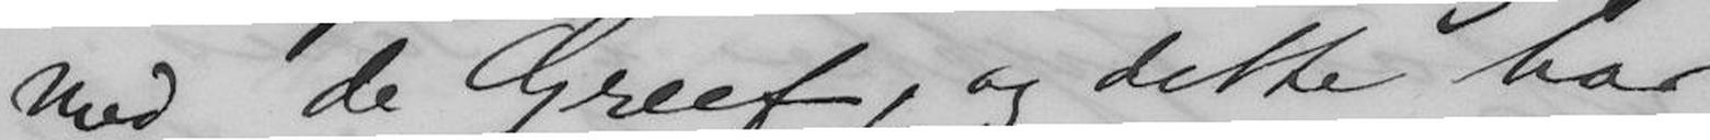med de Greef, og dette har
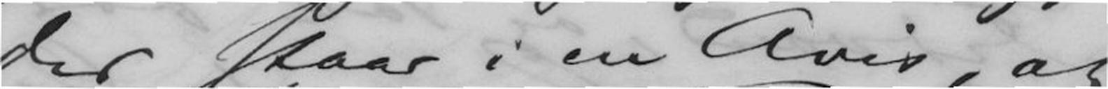der staar i en Avis, at
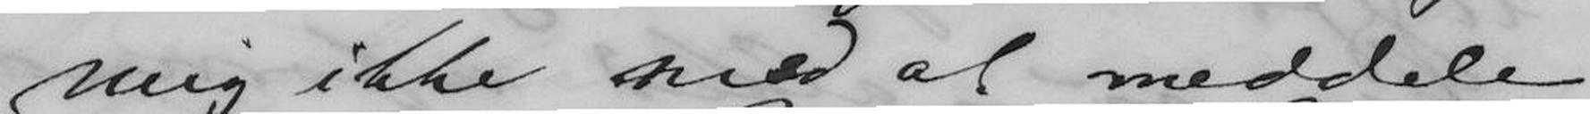mig ikke med at meddele
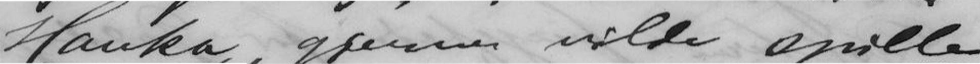Hanka gjerne vilde spille
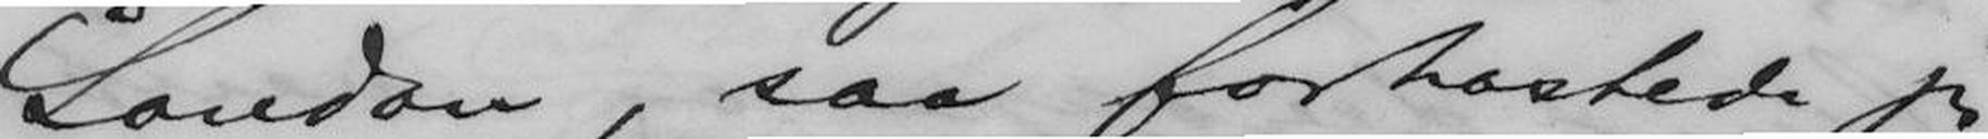London, saa forhastede jeg
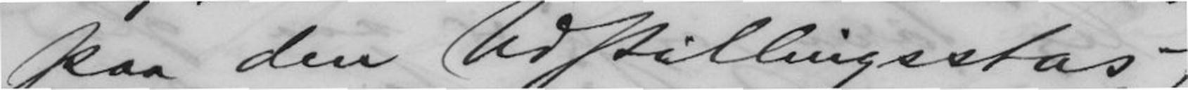paa den Udstillingsstas,
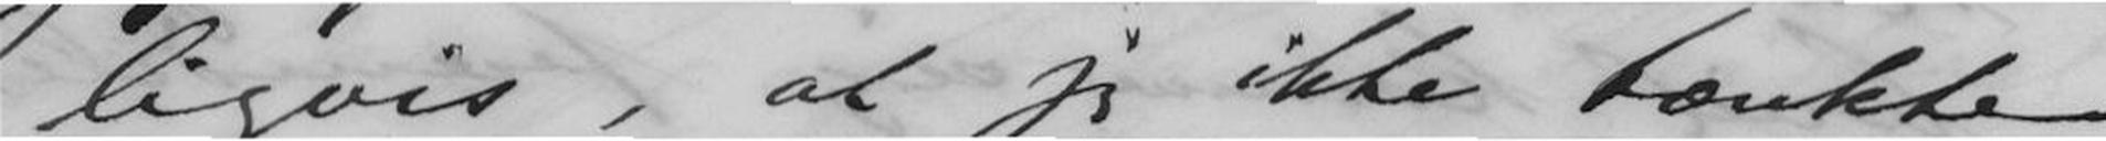ligvis, at jeg ikke tænkte
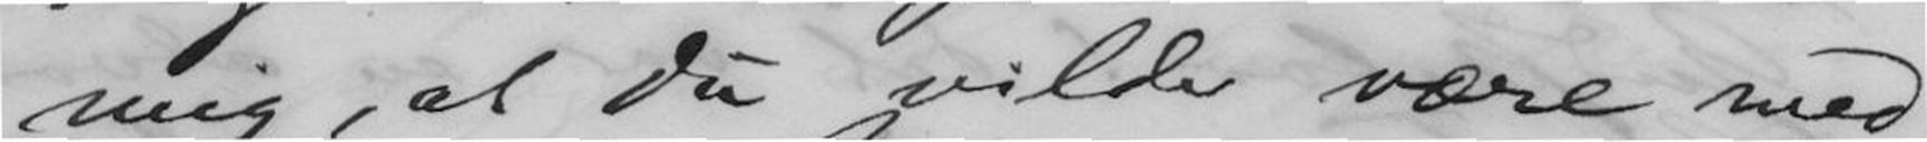mig, at Du vilde være med
